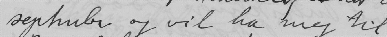september og vil ha meg til
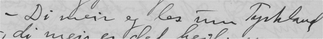– Di meir eg les um Tyskland
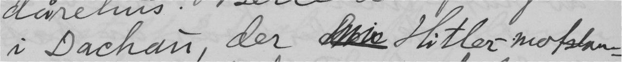i Dachau, der  Hitler-motstan-
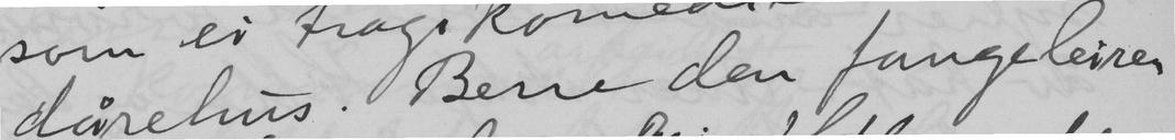dårehus. Berre den fangeleiren
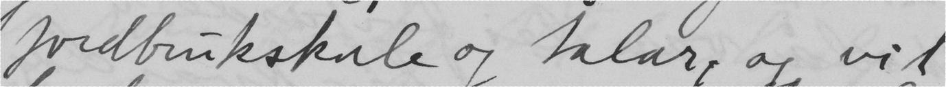jordbrukskule og talar, og vil
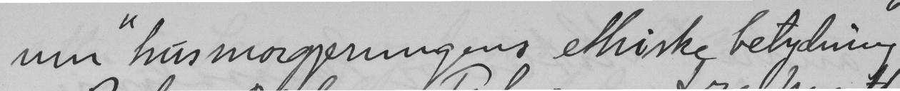um "husmorgjerningens ethiske betydning"
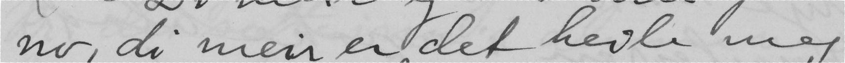no, di meir er det heile meg
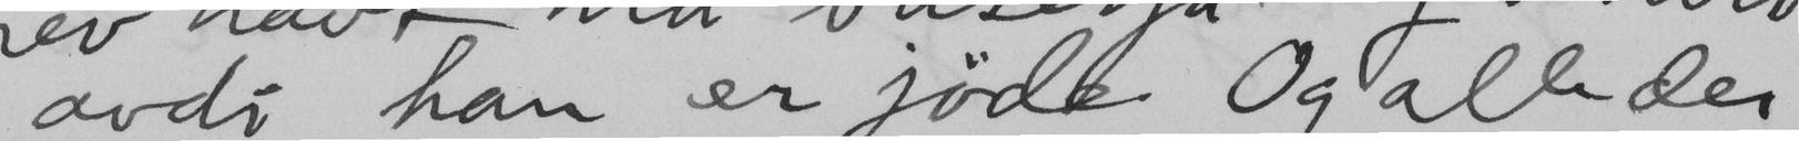avdi han er jöde. Og alle der
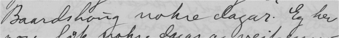Baardshoug nokre dagar. Eg hev
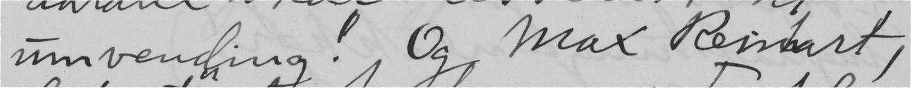umvending! Og Max Reinhart,
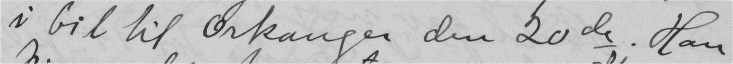i bil til Orkanger den 20de. Han
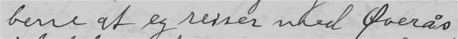berre at eg reiser med Øverås
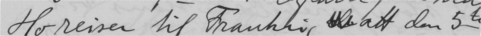Ho reiser til Frankrig att den 5te
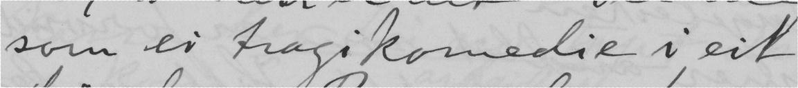som ei tragikomedie i eit
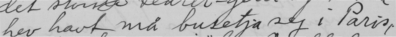hev havt må busetja seg i Paris,
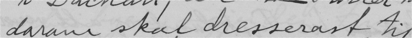darane skal dresserast til
